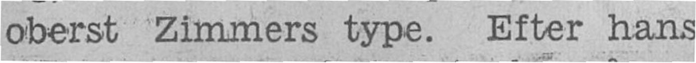oberst Zimmers type. Efter hans
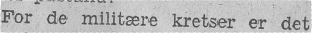For de militære kretser er det
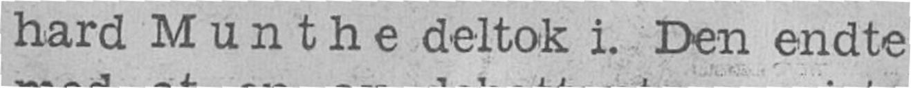hard Munthe deltok i. Den endte
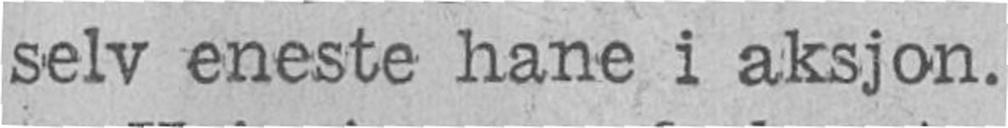selv eneste hane i aksjon.
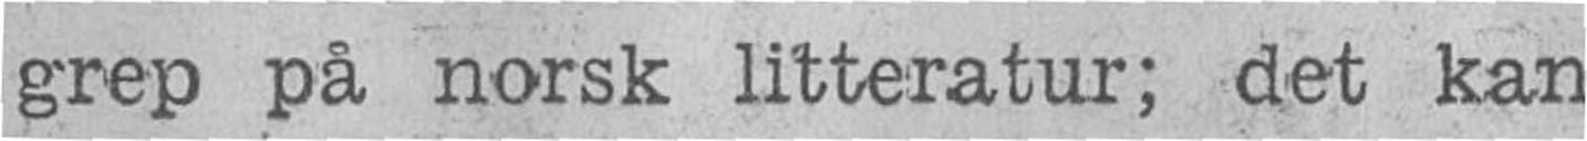grep på norsk litteratur; det kan
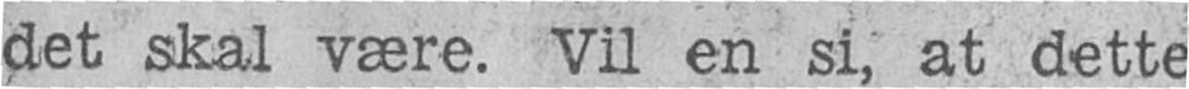det skal være. Vil en si, at dette
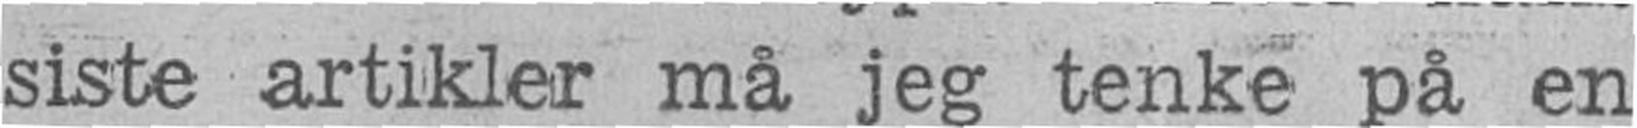siste artikler må jeg tenke på en
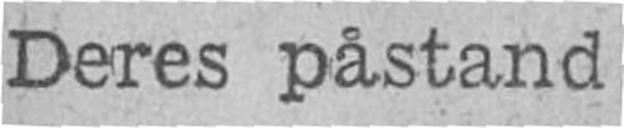Deres påstand!
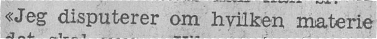«Jeg disputerer om hvilken materie
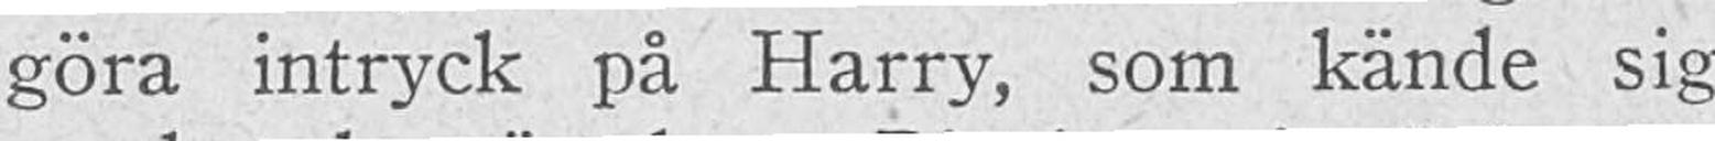göra intryck på Harry, som kände sig
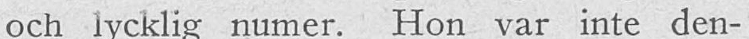och lycklig numer. Hon var inte den-
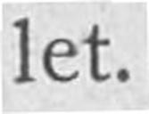let.
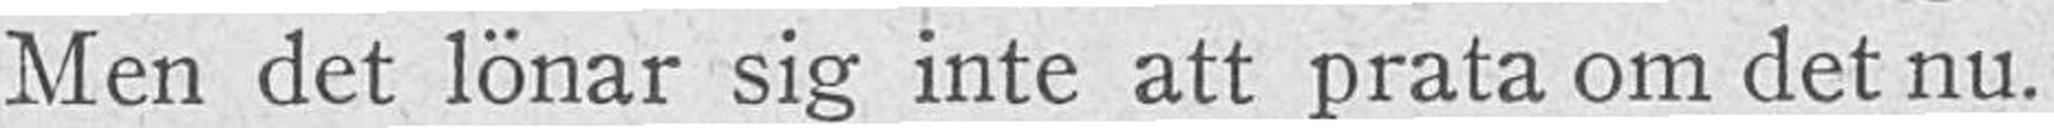Men det lonar sig inte att prata om det nu.
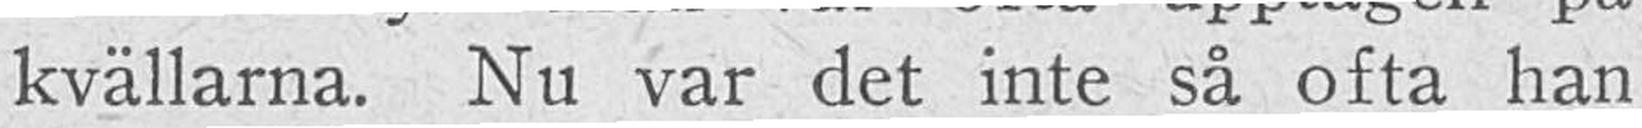kvällarna. Nu var det inte så ofta han
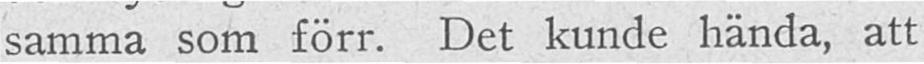samma som förr. Det kunde hända, att
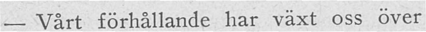— Vårt förhällande har växt oss över
